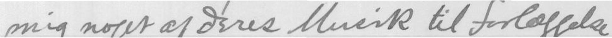mig noget af Deres Musik til Forlæggelse
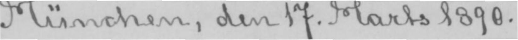München, den 17. Marts 1890.
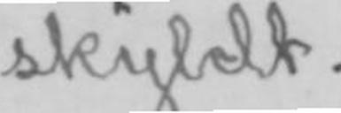skyldt.
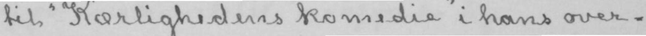til «Kærlighedens komedie» i hans over-
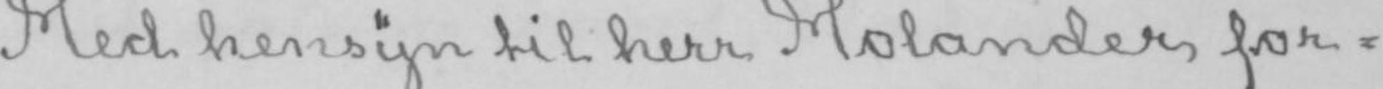Med hensyn til herr Molander for-
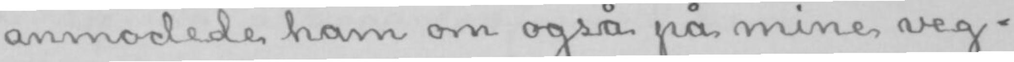anmodede ham om også på mine veg-
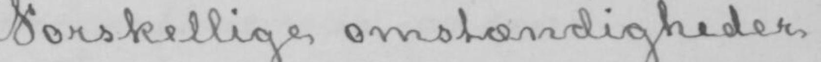Forskellige omstændigheder
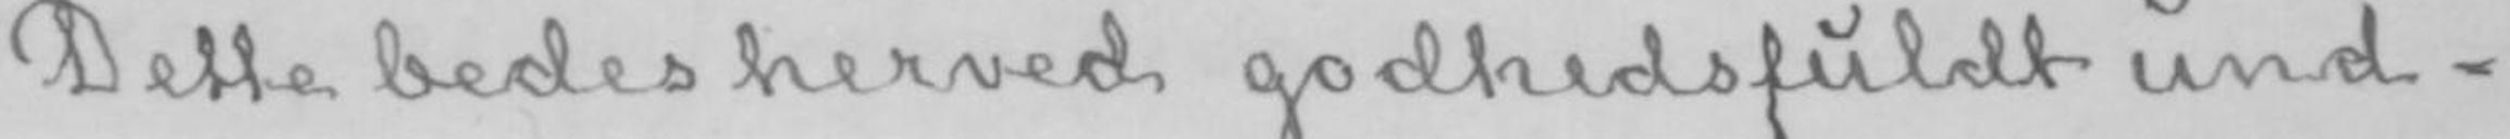Dette bedes herved godhedsfuldt und-
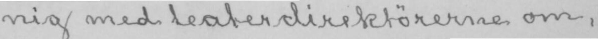nig med teaterdirektørerne om,
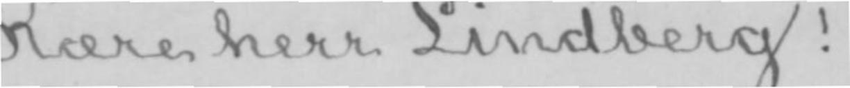Kære herr Lindberg!
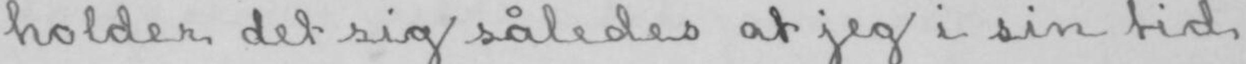holder det sig således at jeg i sin tid
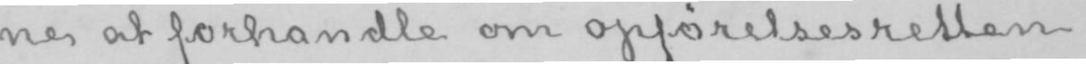ne at forhandle om opførelsesretten
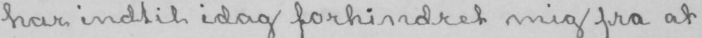har indtil idag forhindret mig fra at
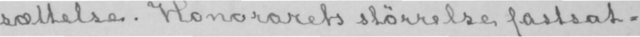sættelse. Honorarets størrelse fastsat-
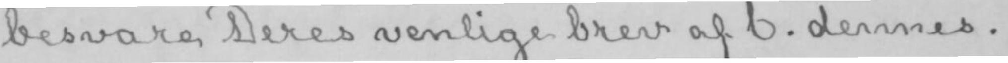besvare Deres venlige brev af 6. dennes.
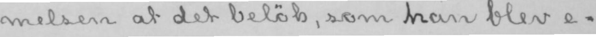melsen at det beløb, som han blev e-
